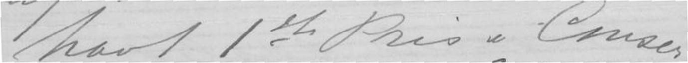havt 1ste Pris i Conser-
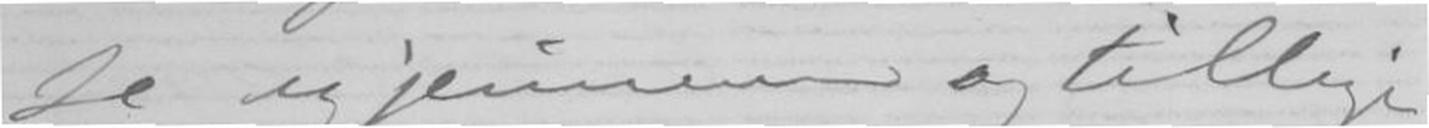se igjennem og tillige
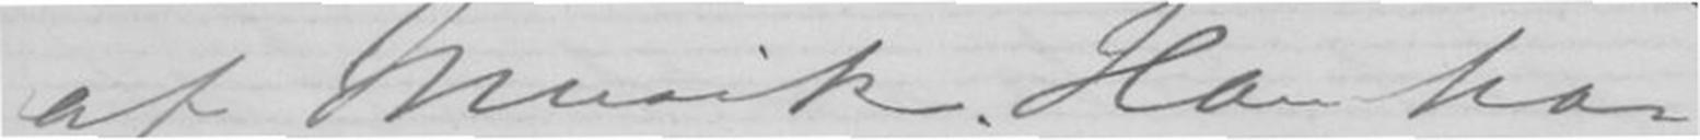af Musik. Han har
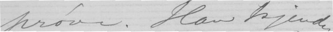prøve. Han kjender
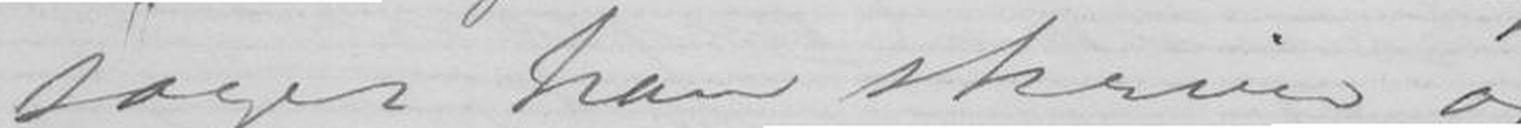sager han skriver og
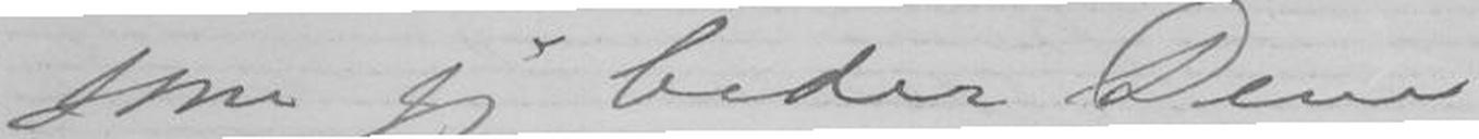som jeg beder Dem
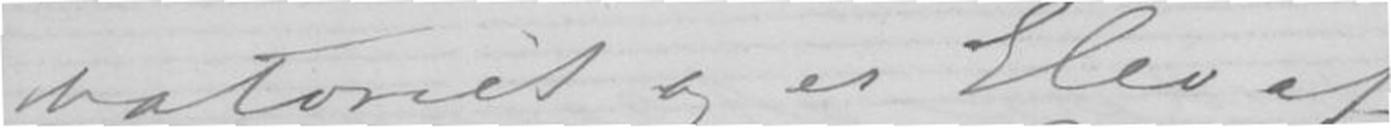vatoriet og er Elev af
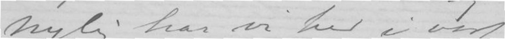nylig har vi her i vort
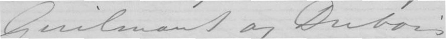Gerilmont og Dubois.
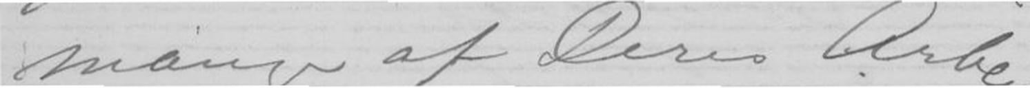mange af Deres Arbeide
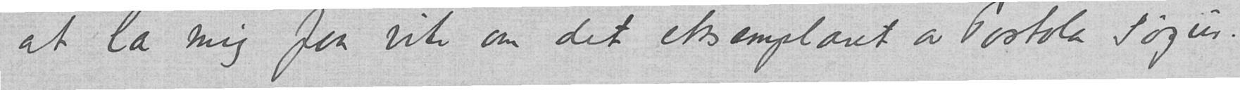at la mig faa vite om det eksemplaret av Postola Sogur.
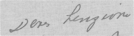Deres hengivne
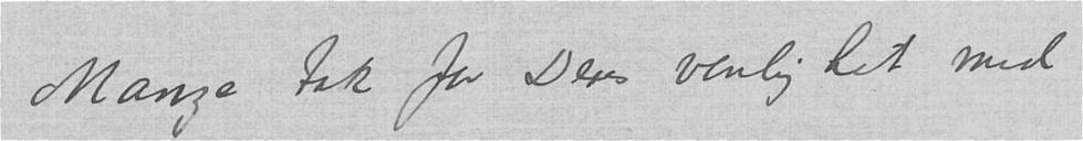Mange tak for Deres venlighet med
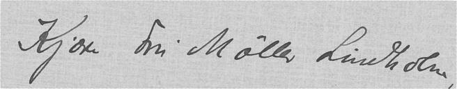Kjære Fru Møller Lindholm,
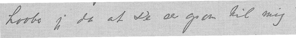haaber jeg da at De ser opom til mig?
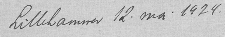Lillehammer 12. mai 1924.
